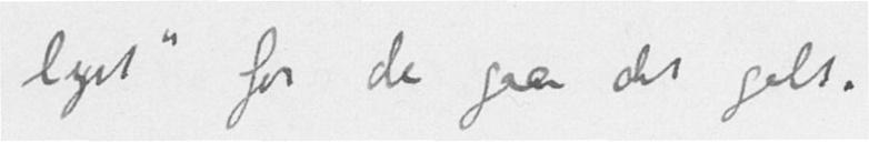lyst" for da gaar det galt.
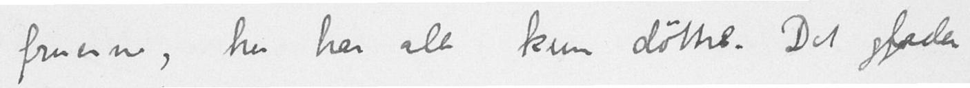fruerne, her har alle kun døttre. Det glæder
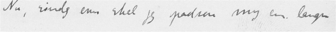Nu, søndag enn skal jeg spadsere mig en længre
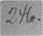246.
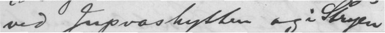ved Jupvashytten og i Stryen,
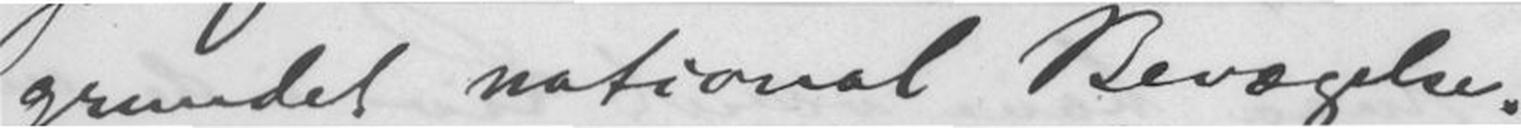grundet national Bevægelse.
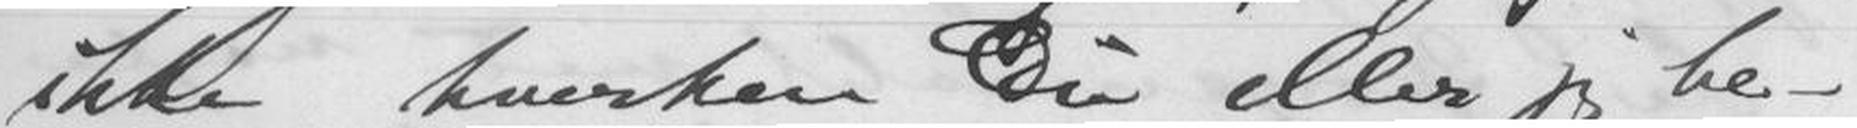ikke hverken Du eller jeg be-
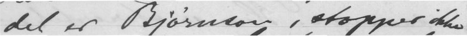det er Bjørnson, stopper ikke
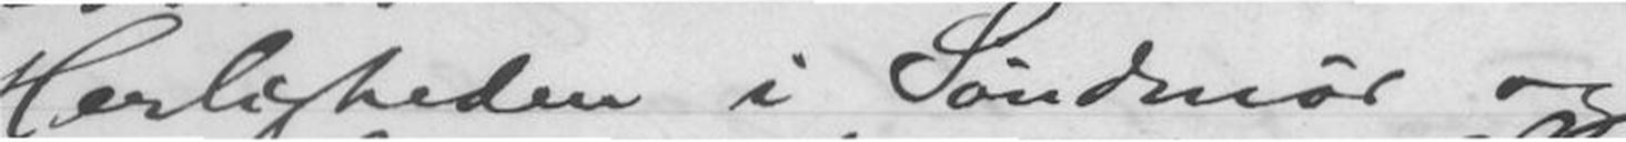Herligheden i Søndmør og
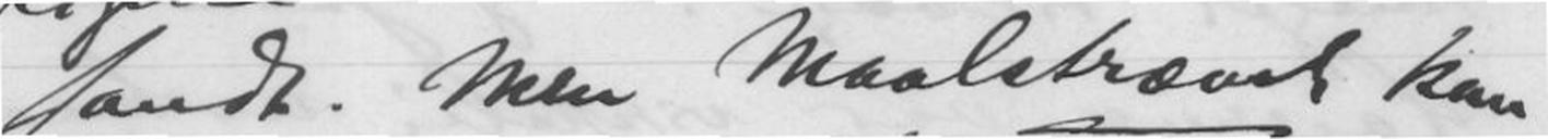sandt. Men Maalstrævet kan
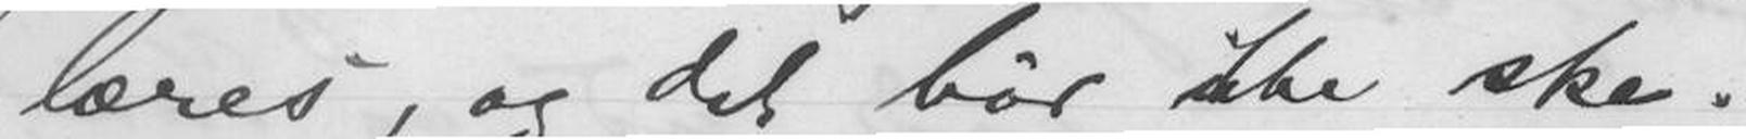læres, og det bør ikke ske.
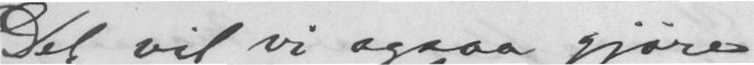Det vil vi ogsaa gjøre
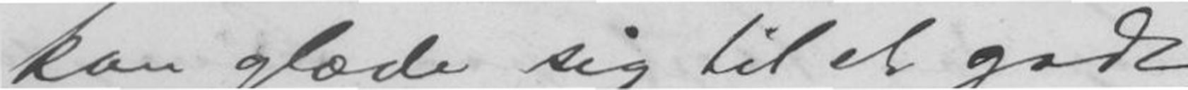kan glæde sig til et godt
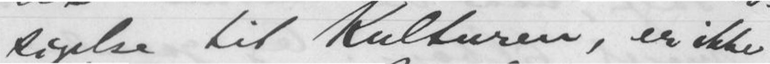sigelse til Kulturen, er ikke
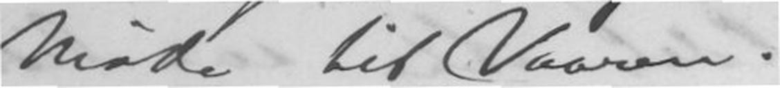møde til Vaaren.
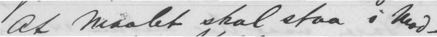At Maalet skal staa i Mod-
